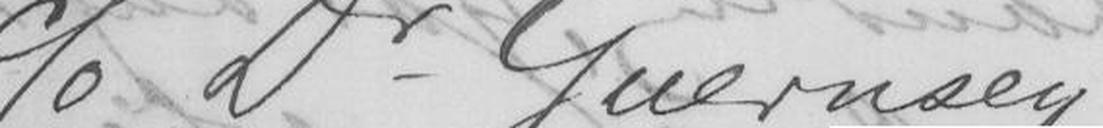c/o Dr Guernsey
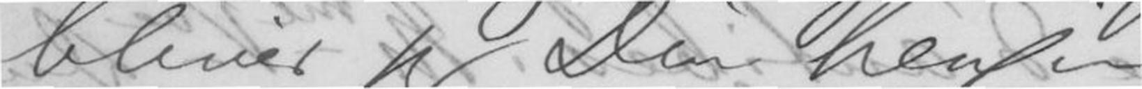bliver jeg Din Hengivne
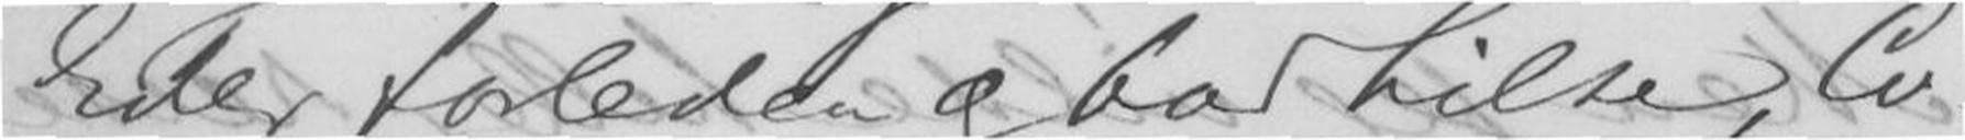Eder forleden og bad hilse, Co-
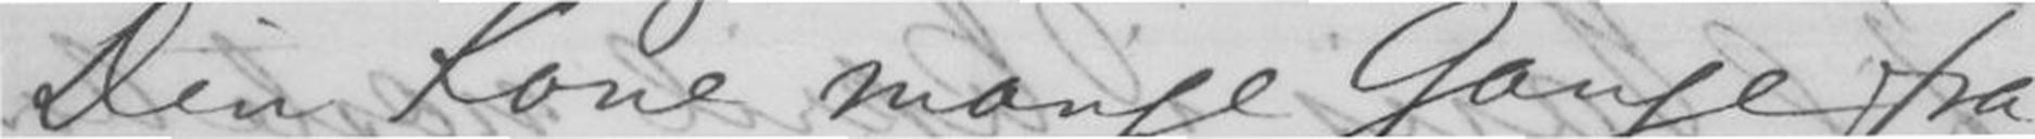Din Kone mange Gange fra
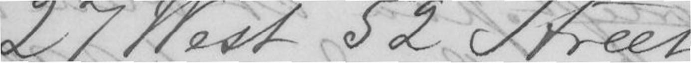27 West 52 Street
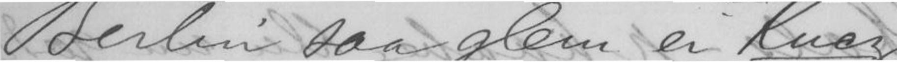Berlin saa glem ei Kuez
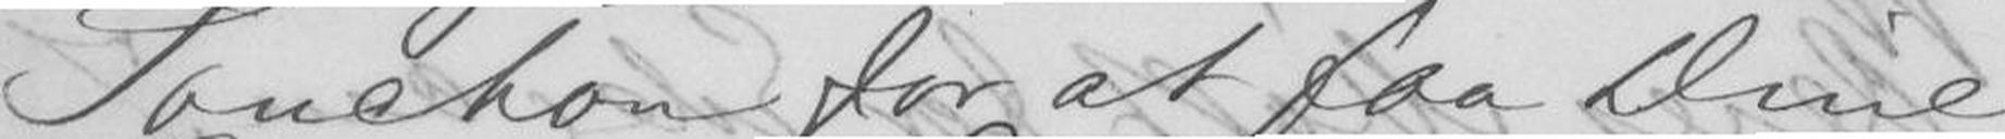Souchou for at faa Dine
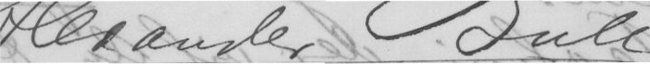Alexander Bull
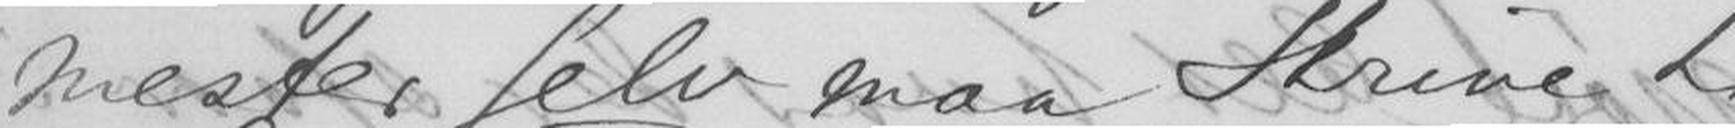mester selv maa skrive til
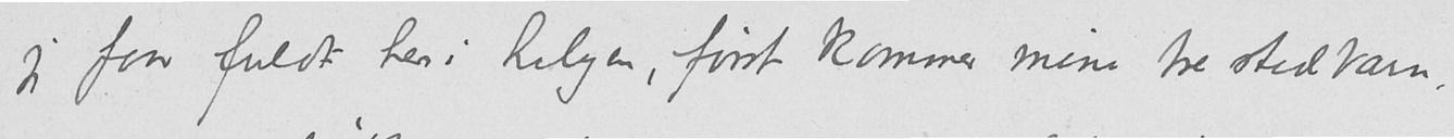jeg faar fuldt her i helgen, først kommer mine tre stedbarn,
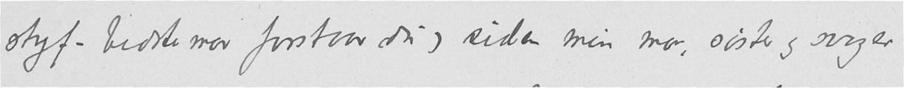styf-bedstemor forstaar du) siden min mor, søster og svoger
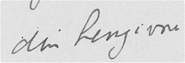din hengivne
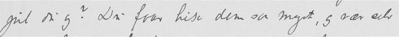jul du og? Du faar hilse dem saa meget, og vær selv
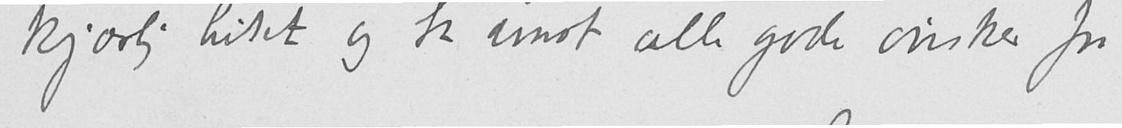kjærlig hilset og ta imot alle gode ønsker fra
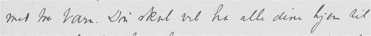med tre barn. Du skal vel ha alle dine hjem til
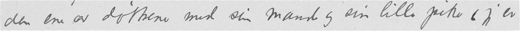den ene av døttrene med sin mand og sin lille pike (jeg er
